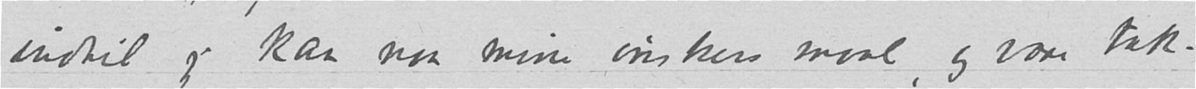indtil jeg kan naa mine ønskers maal, og være tak-
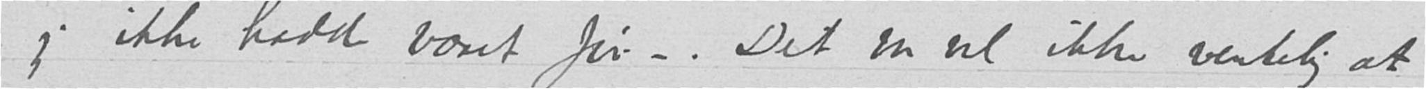jeg ikke hadde været før –. Det var vel ikke ventelig at
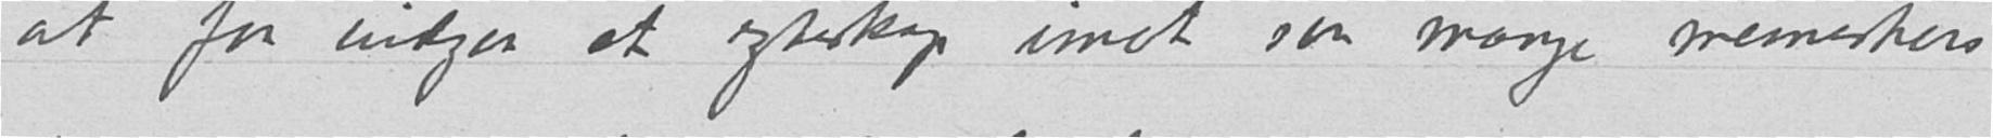at faa indgaa et egteskap imot saa mange menneskers
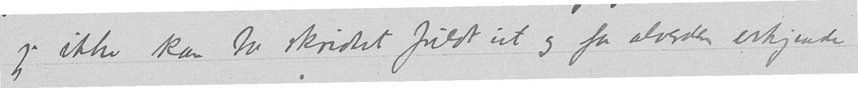jeg ikke kan ta skridtet fuldt ut og for alverden erkjende
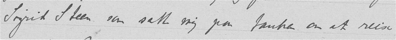Sigrid Steen som satte mig paa tanken om at reise
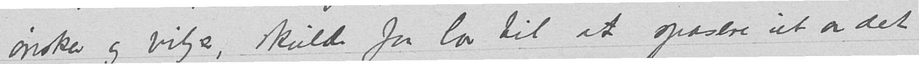ønsker og vilje, skulde faa lov til at spasere ut av det
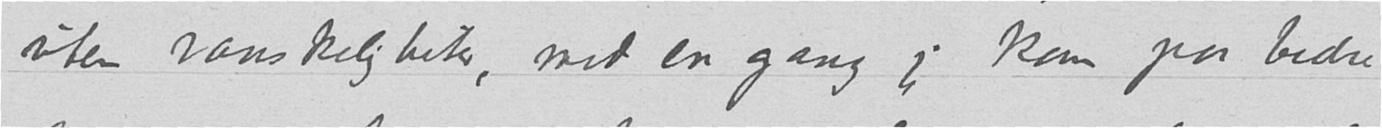uten vanskeligheter, med en gang jeg kom paa bedre
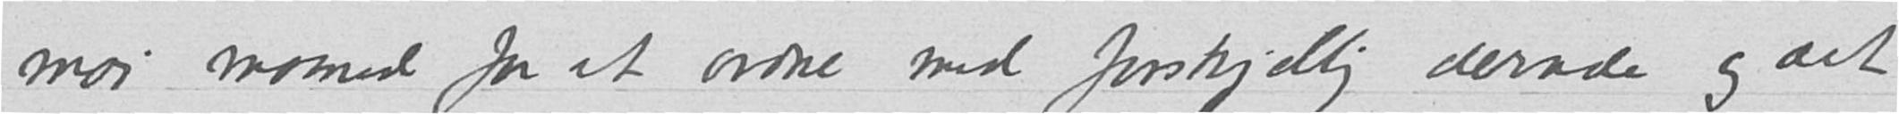mai maaed for at ordne med forskjellig derude og det
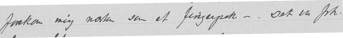forekom mig næsten som et fingerpæk –. Det var frk.
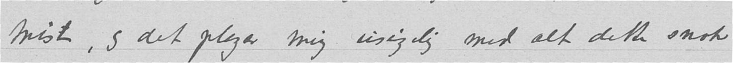trist, og det plager mig usigelig med alt dette snak
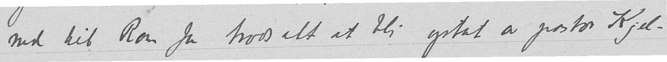ned til Rom for trods alt at bli optat av pastor Kjel-
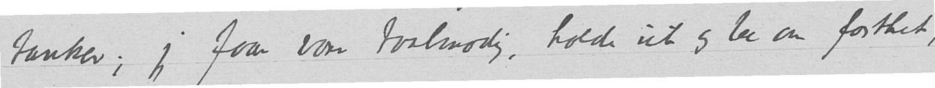tanker; jeg faar være taalmodig, holde ut og be om fasthet,
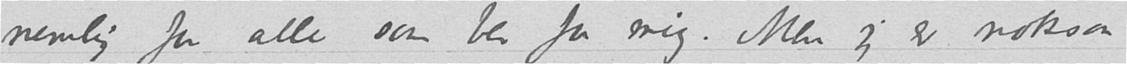nemlig for alle som ber for mig. Men jeg er noksaa
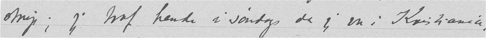strup; jeg traf hende i søndags da jeg var i Kristiania,
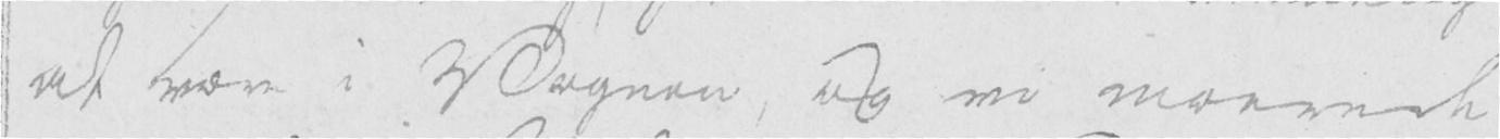at være i Vognen, og vi moerede
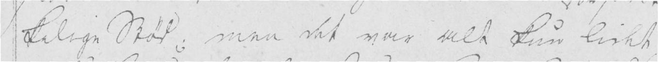kelige Stød; men det var alt kun lidet
